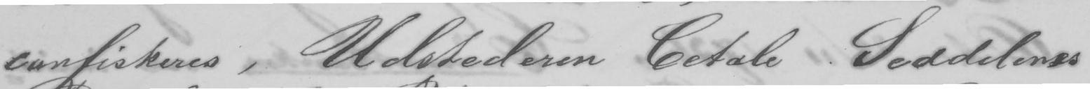confiskeres, Udstederen betale Seddelenes
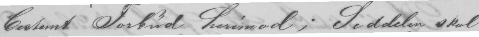bestemt Forbud herimod; Seddelen skal
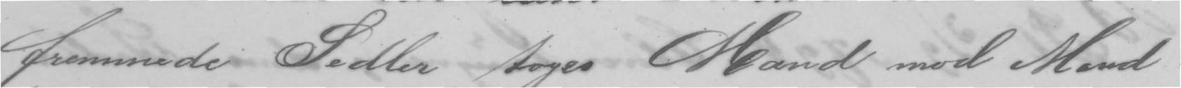fremmede Sedler tages Mand mod Mand
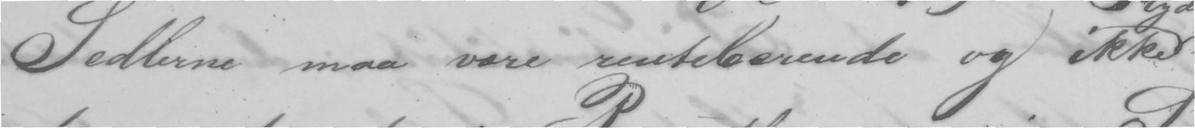Sedlerne maa være rentebærende og ikke
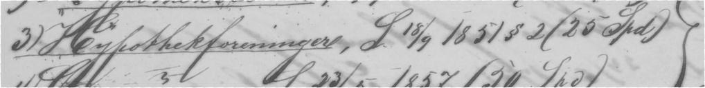3) Hypothtekforeninger, L. 18/9 1851 § 2 (25 Spd)
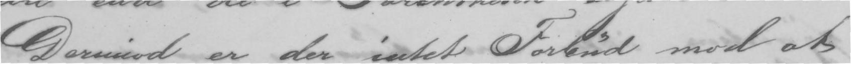Derimod er der intet Forbud mod at
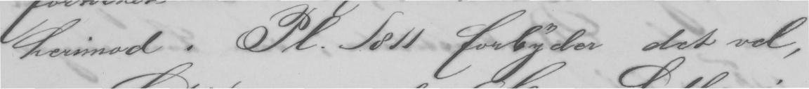herimod. Pl. 1811 forbyder det vel,
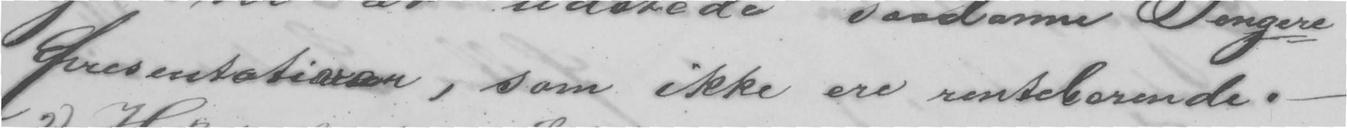Presentationen, som ikke ere rentebærende. -
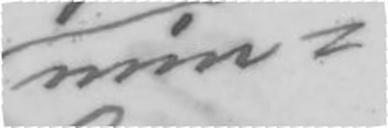min-
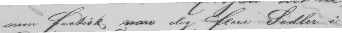men faktisk vare dog flere Sedler i
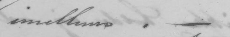imellem. -
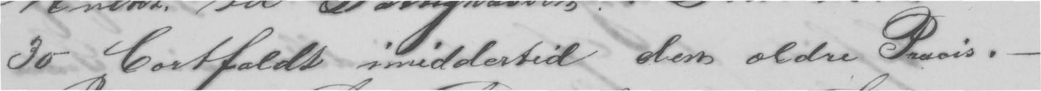30 bortfaldt imidlertid den ældre Pracis. -
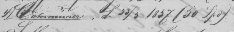4) Communen, L 23/5 1857 (50 Spd)
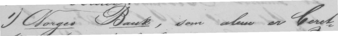1) Norges Bank, som alene er beret-
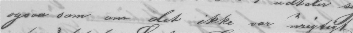ogsaa som om det ikke var urigtigt
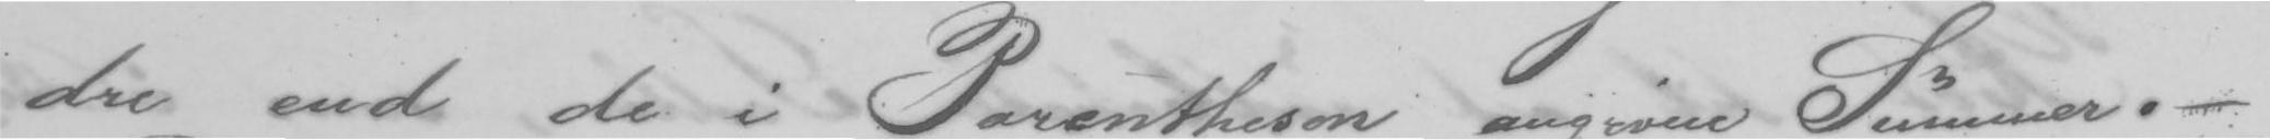dre end de i Parenthesen angivne Summer. -
